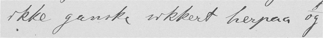ikke ganske sikkert herpaa og
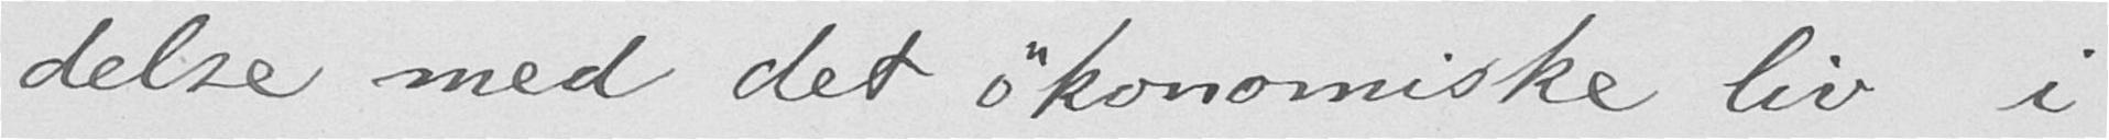delse med det økonomiske liv i
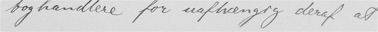boghandlere for uafhængig deraf at
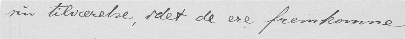sin tilværelse, idet de ere fremkomne
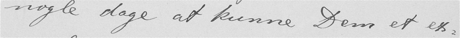nogle dage at kunne Dem et ex-
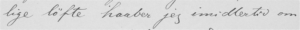lige løfte haaber jeg imidlertid om
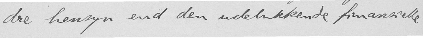dre hensyn end den udelukkende finansielle
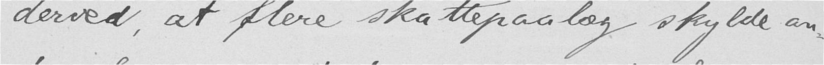derved, at flere skattepaalæg skylde an-
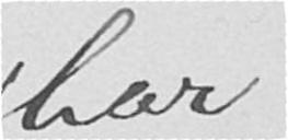har
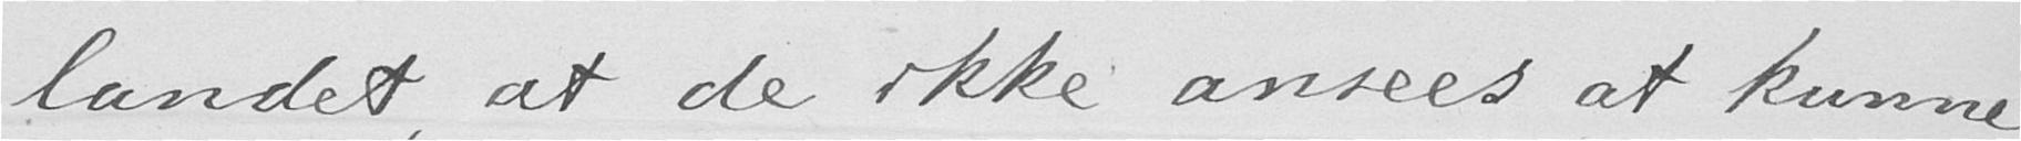landet, at de ikke ansees at kunne
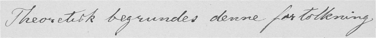Theoretisk begrundes denne fortolkning
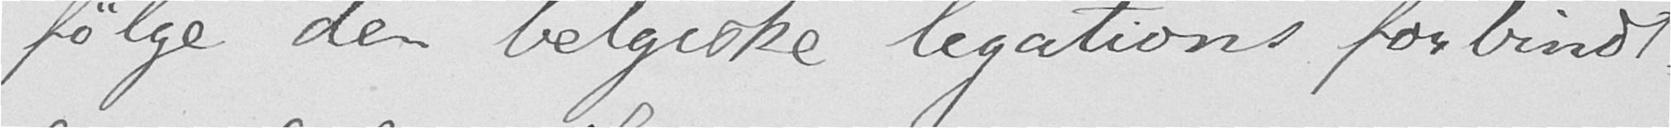følge den belgiske legations forbindt-
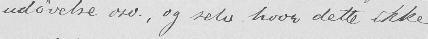udøvelse osv., og selv hvor dette ikke
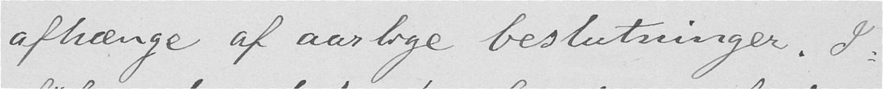afhænge af aarlige beslutninger. I-
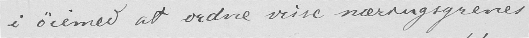i øiemed at ordne visse næringsgrenes
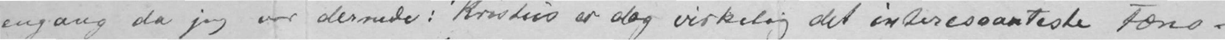engang da jeg var dernede: «Kristus er dog virkelig det interessanteste Fæno-
